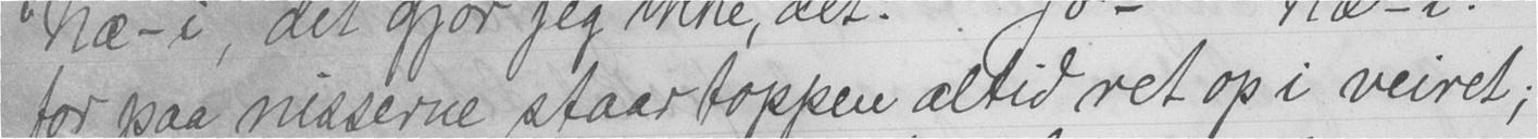for paa nisserne staar toppen altid ret op i veiret;
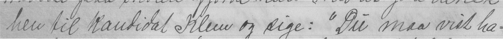hen til kandidat Klem og sige: "Du maa vist ha-
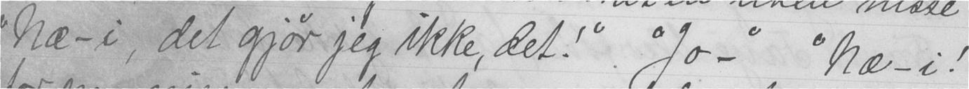"Næ-i, det gjør jeg ikke, det!" "Jo-" "Næ-i!
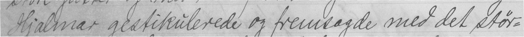Hjalmar gestikulerede og fremsagde med det stør-
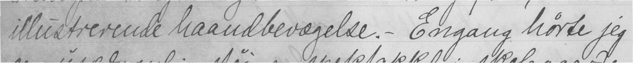illustrerende haandbevægelse. - Engang hørte jeg
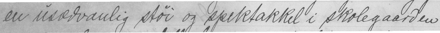en usædvanlig støi og spektakkel i skolegaarden
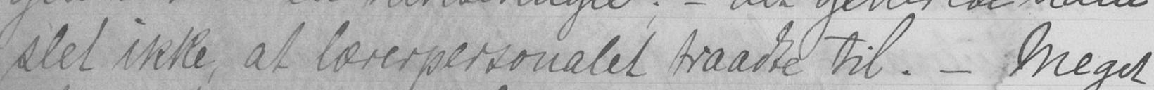slet ikke, at lærerpersonalet traadte til. - Meget
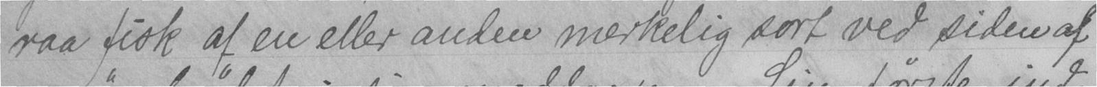raa fisk af en eller anden merkelig sort ved siden af
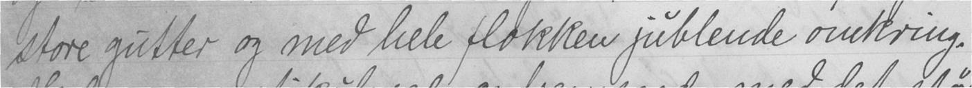store gutter og med hele flokken jublende omkring.
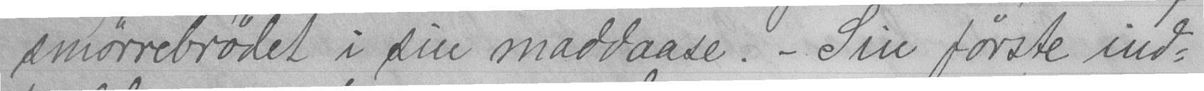smørrebrødet i sin maddaase. - Sin første ind-
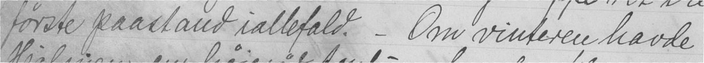første paastand iallefald. - Om vinteren havde
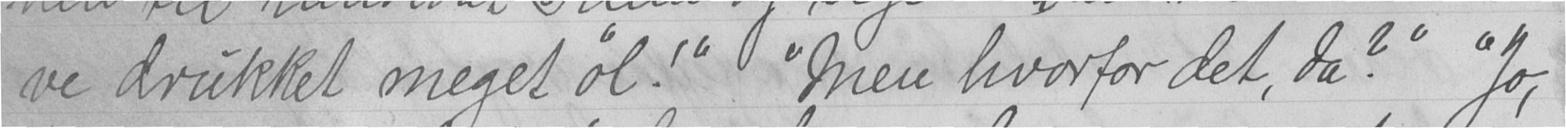ve drukket meget øl!" "Men hvorfor det, da?" "Jo,
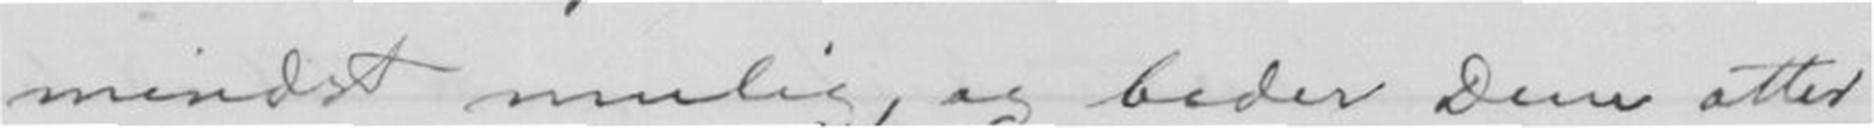mindst mulig, og beder Dem atter
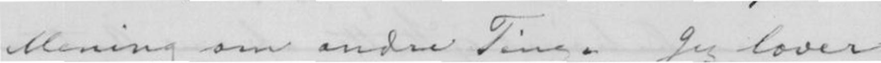Mening om andre Ting. Jeg lover
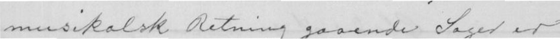musikalsk Retning gaaende Sager er
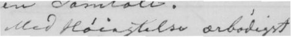Med høiagtelse ærbødigst
